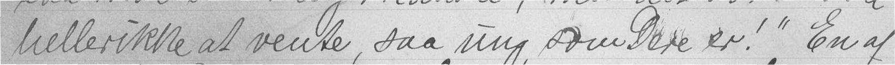hellerikke at vente, saa ung, som Dere er!" En af
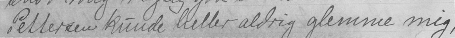Pettersen kunde heller aldrig glemme mig,
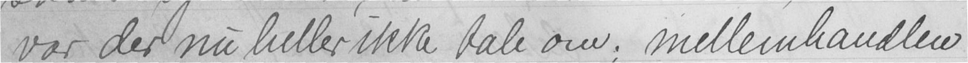var der nu heller ikke tale om; mellemhandlen
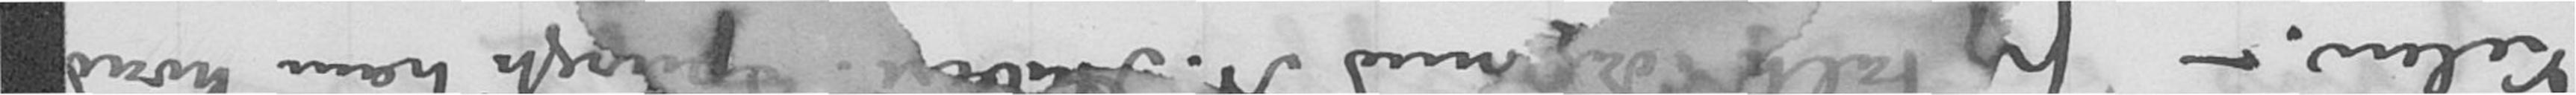kelen. - Jeg talte da med A. Aubert. Spurgte ham hvad
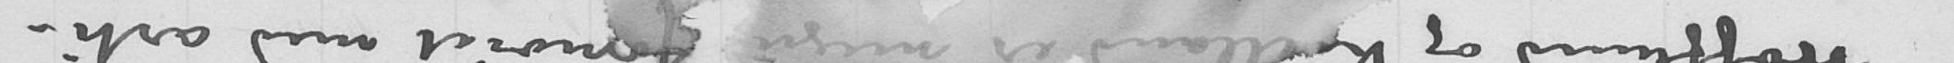Hofflund og Kielland er meget fornøiet med arti-
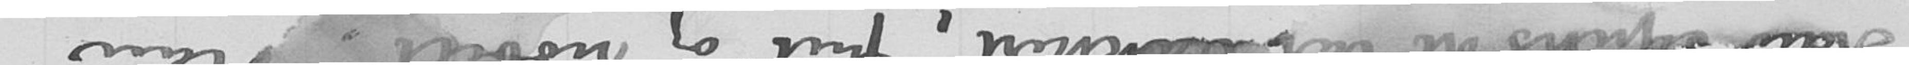Han syntes det var "vakkert, fint og nobelt“. Han
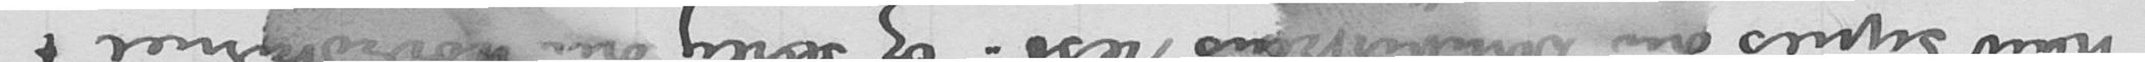han synes om Domkirkens rest. og særlig om hovedtårnet.
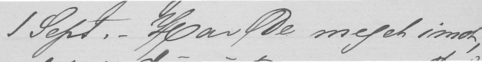i Sept. Har De meget imot,
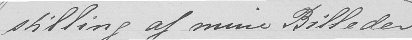stilling af mine Billeder
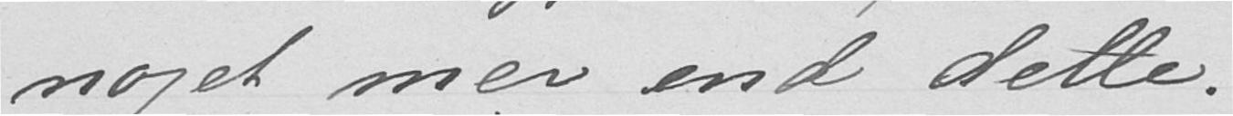noget mer end dette.
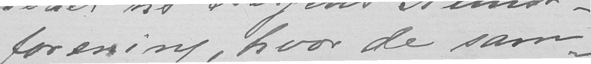forening, hvor de sam-
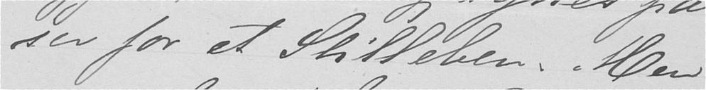ser for et Stilleben. Men
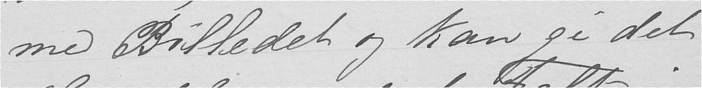med Billedet og kan gi det
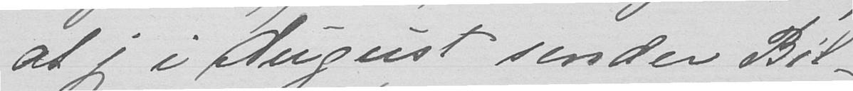at jeg i August sender Bil-
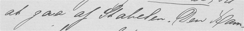at gaa af Stabelen. Den Ram-
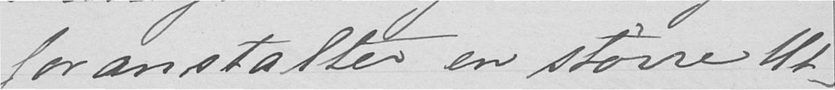foranstalter en større Ut-
